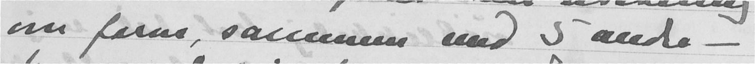om form, sammen med 5 andre -
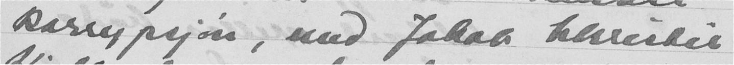kasseopsjon med Jakob Christie
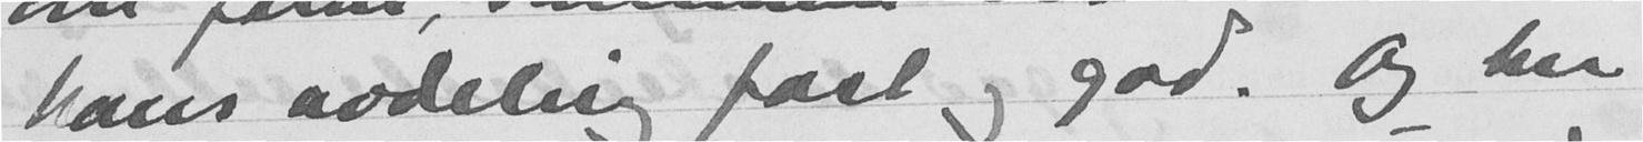hans avdeling fast og god. Og her
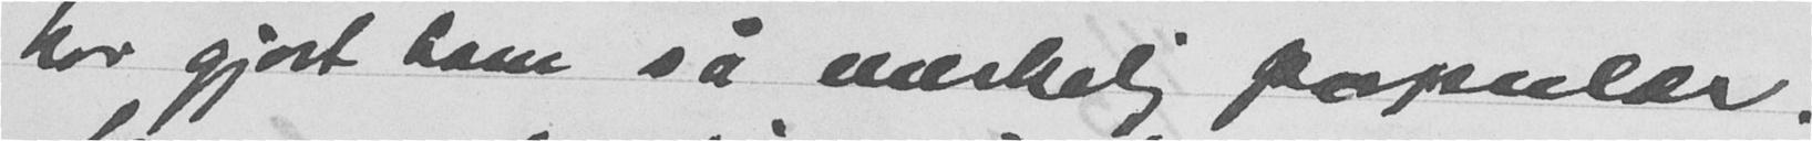har gjort ham sa merkelig populær.
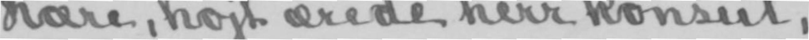Kære, højt ærede herr konsul,
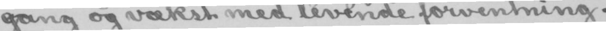gang og vækst med levende forventning.
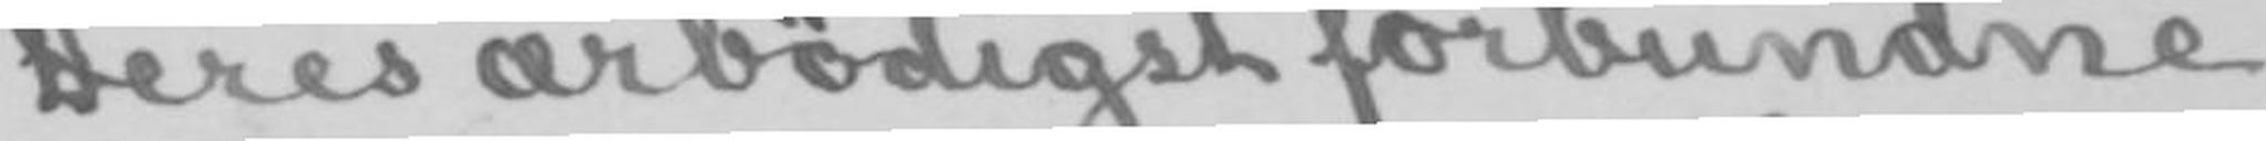Deres ærbødigst forbundne
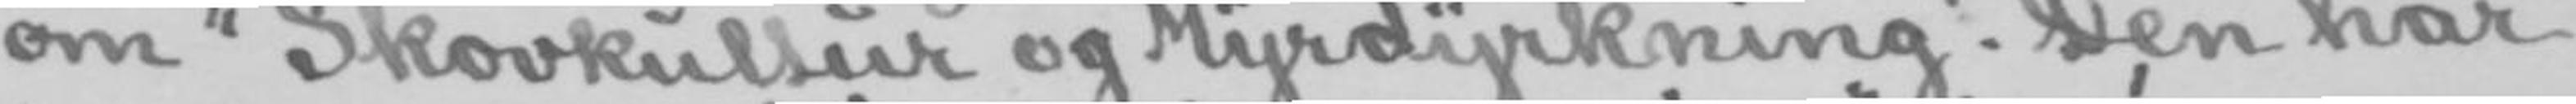om «Skovkultur og Myrdyrkning». Den har
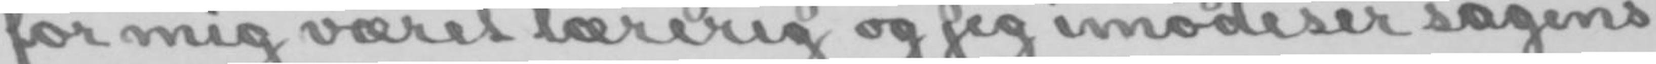for mig været lærerig og jeg imødesér sagens
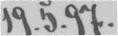19.5.97.
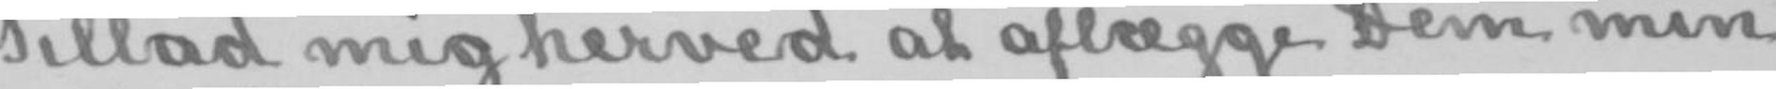Tillad mig herved at aflægge Dem min
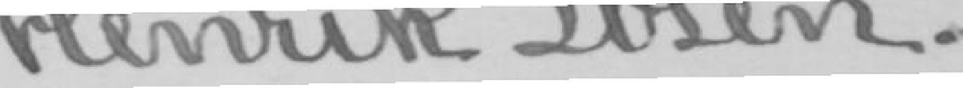Henrik Ibsen.
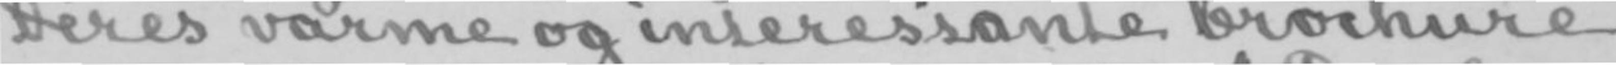Deres varme og interessante brochure
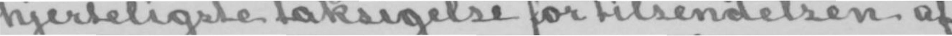hjerteligste taksigelse for tilsendelsen af
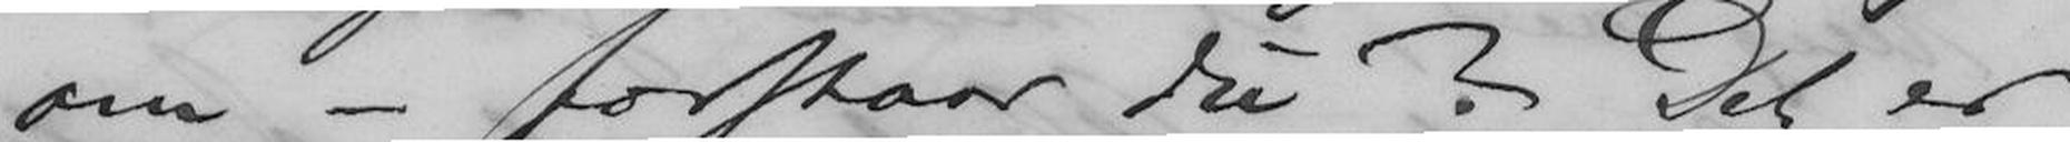om - forstaar du? Det er
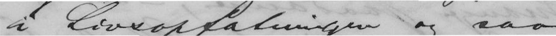i Livsopfatningen og saa
